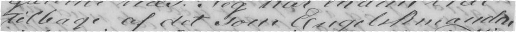tilbage af det som Engelskmanden
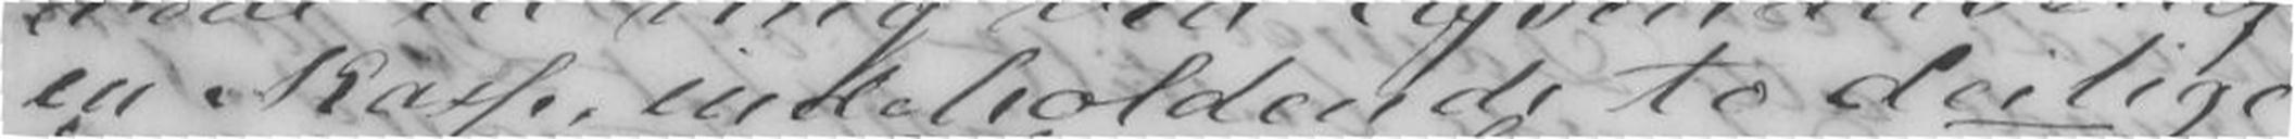en Kasse, indeholdende to deilige
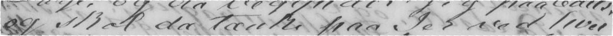og skal da tænke paa Jer ved hver
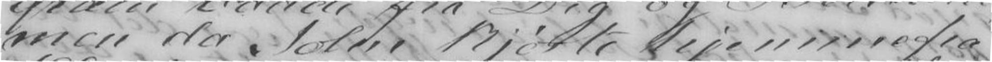men da John kjørte hjemmefra
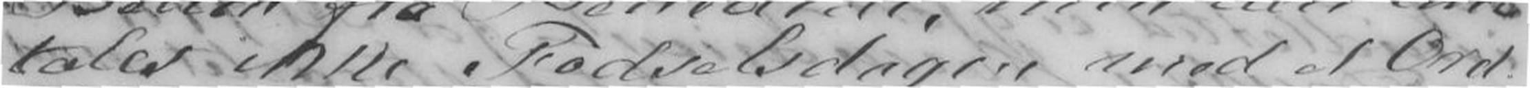tales ikke Fødselsdagen med et Ord.
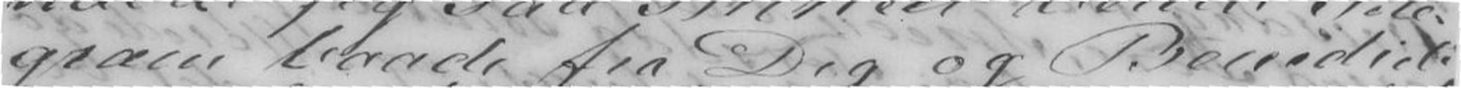gram baade fra Dig og Benedicte,
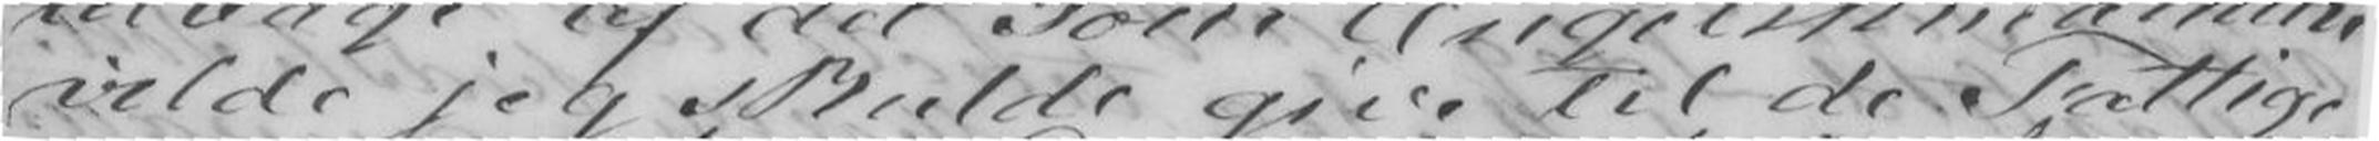vilde jeg skulde give til de Fattige,
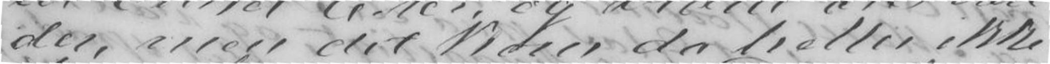der, men det kom da heller ikke
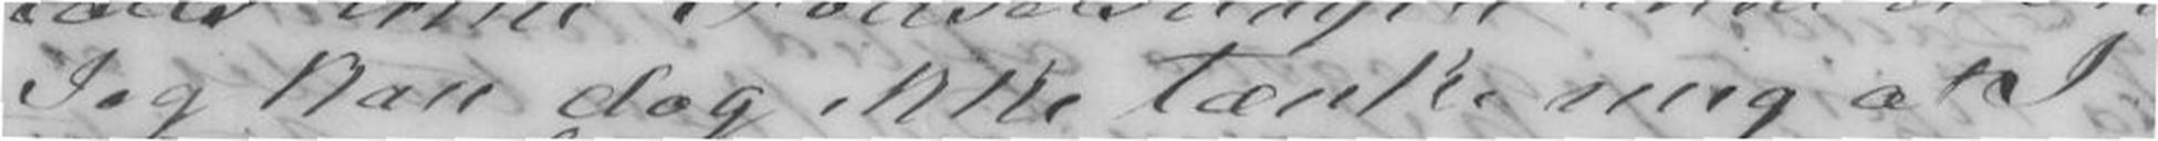Jeg kan dog ikke tænke mig at I
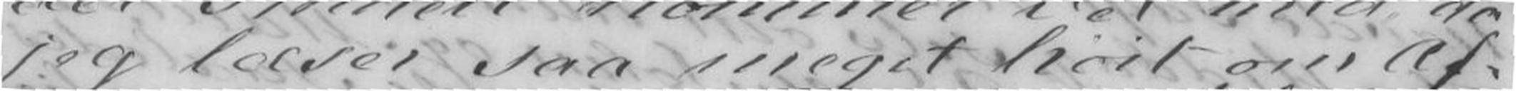jeg læser saa meget høit om Af-
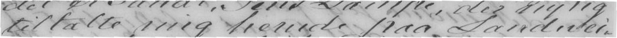tiltalte mig herude paa Landevei-
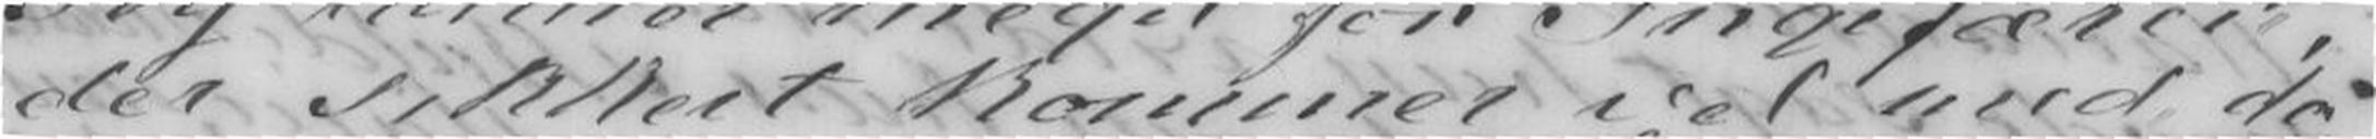der sikkert kommer vel med da
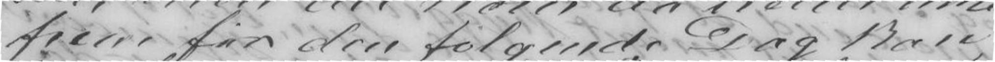frem før den følgende Dag kan
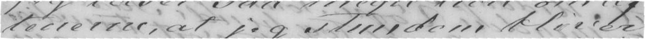tenerne, at jeg stundom bliver
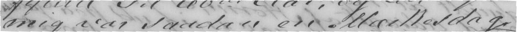mig var saadan en Mærkesdag
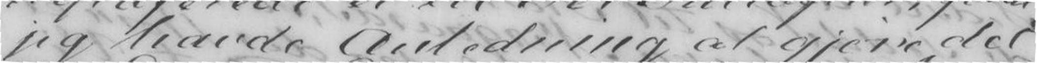jeg havde Anledning at gjøre det
